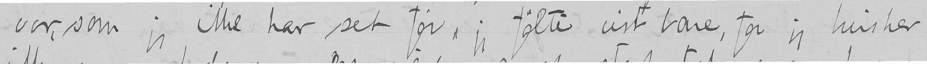vor, som jeg ikke har set før, jeg følte vist bare, for jeg husker
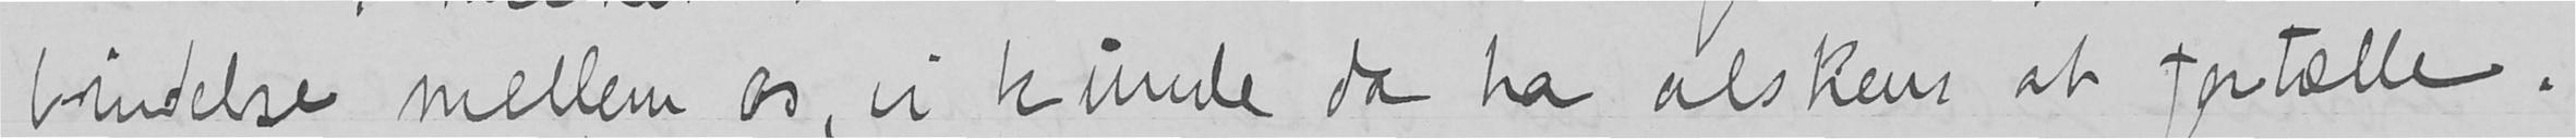bindelse mellem os, vi kunde da ha alskens at fortælle.
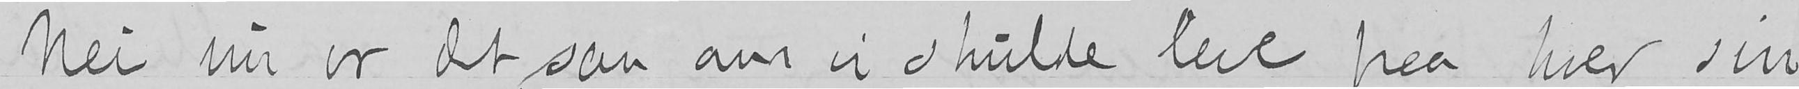Nei nu er det som om vi skulde leve paa hver sin
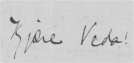Kjære Veda!
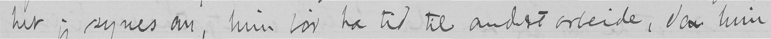ket jeg synes om, hun bør ha tid til andet arbeide, dar hun
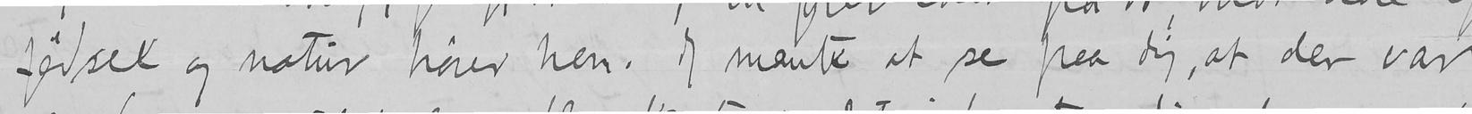fødsel og natur hører hen. Jeg mente at se paa dig, at der var
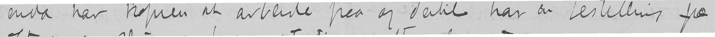enda har kopien at arbeide paa og dertil har en bestilling fra
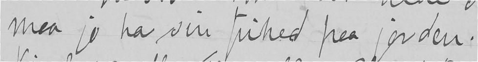maa jo ha sin frihed paa jorden.
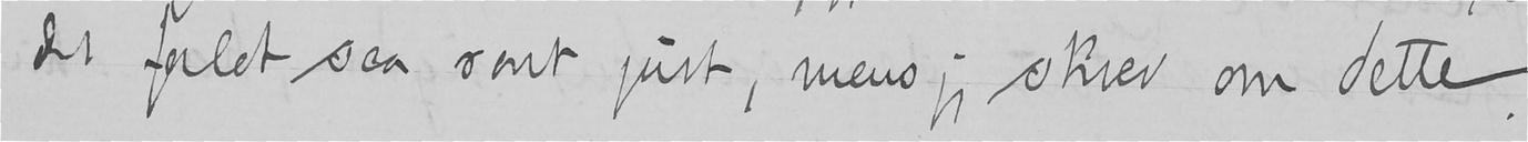det faldt saa sant just, mens jeg skrev om dette.
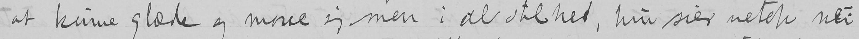at kunne glæde og more sig men i al stilhed, hun sier netop nei
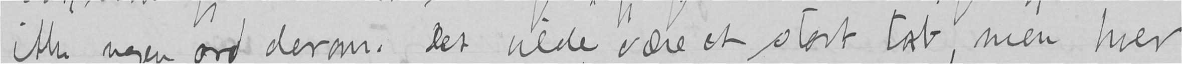ikke nogen ord derom. Det vilde være et stort tab, men hver
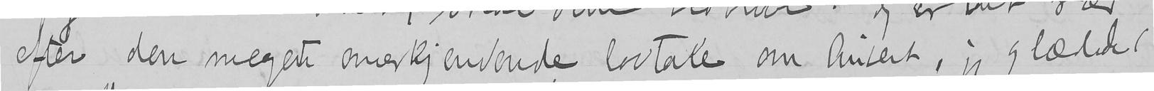efter den meget anerkjendende lovtale om Aubert, jeg glæder
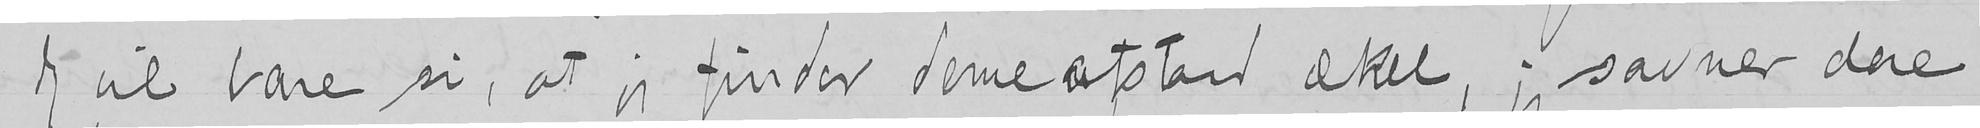Jeg vil bare si, at jeg finder denne afstand ækel, jeg savner dere
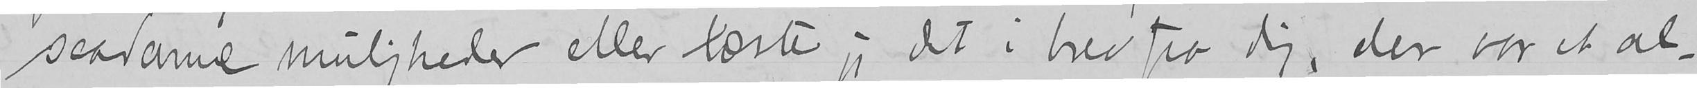saadanne muligheder eller læste jeg det i brev fra dig, der var et al-
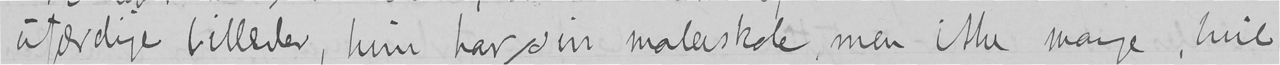ufærdige billeder, hun har sin malerskole, men ikke mange, hvil-
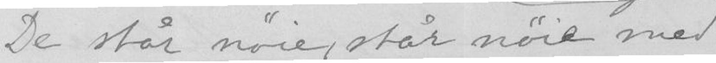De står nøie, står nøie med
